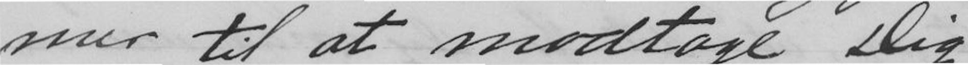mer til at modtage Dig
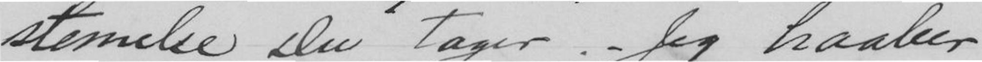stemelse Du tager. - Jeg haaber
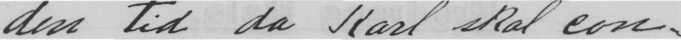den tid da Karl skal con-
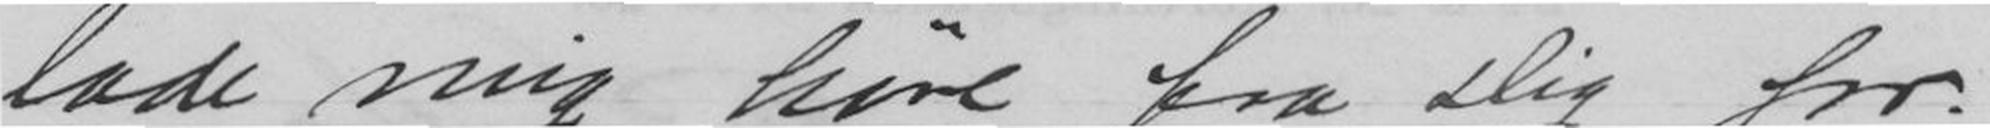lade mig høre fra Dig pr.
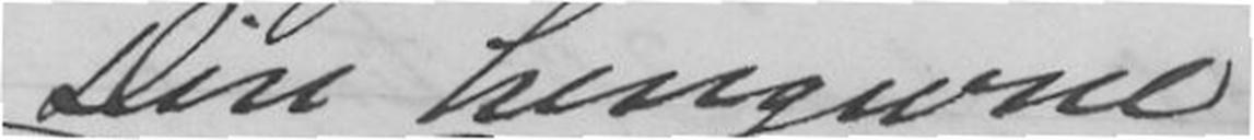Din hengivne
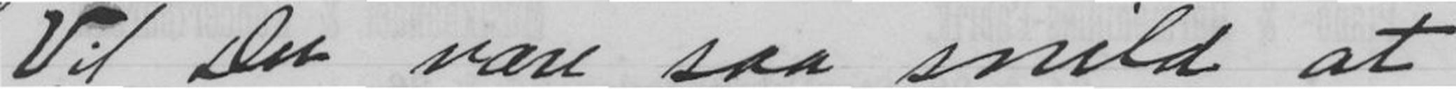Vil Du være saa snild at
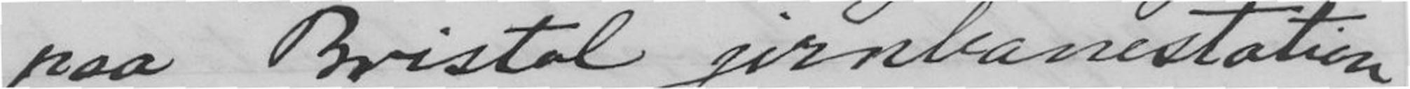paa Bristol jernbanestation
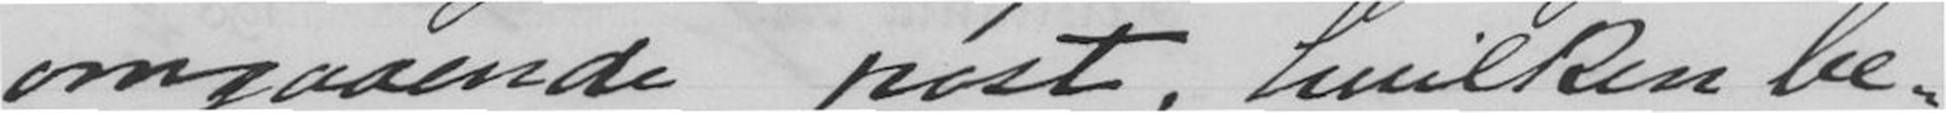omgaaende post, hvilken be-
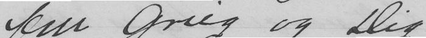fru Grieg og Dig,
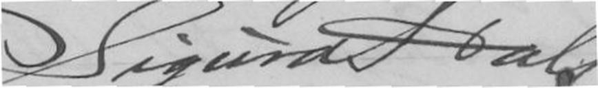Sigurd Hals
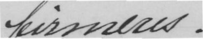firmeres.
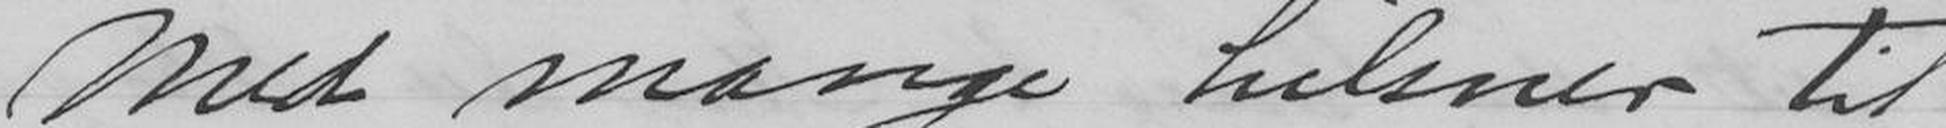Med mange hilsner til
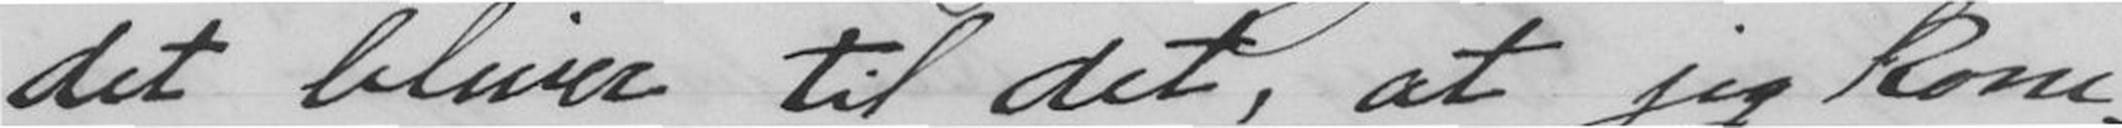det bliver til det, at jeg kom-
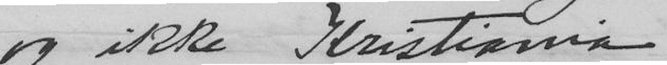og ikke Kristiania
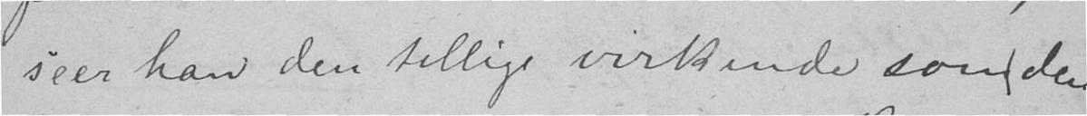seer han den tillige virkende som den
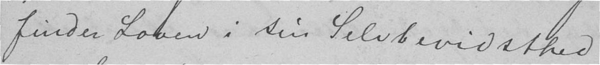finder Loven i sin Selvbevidsthed,
